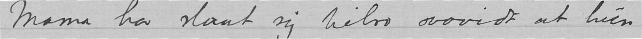Mama har slaat sig tilro saavidt at hun
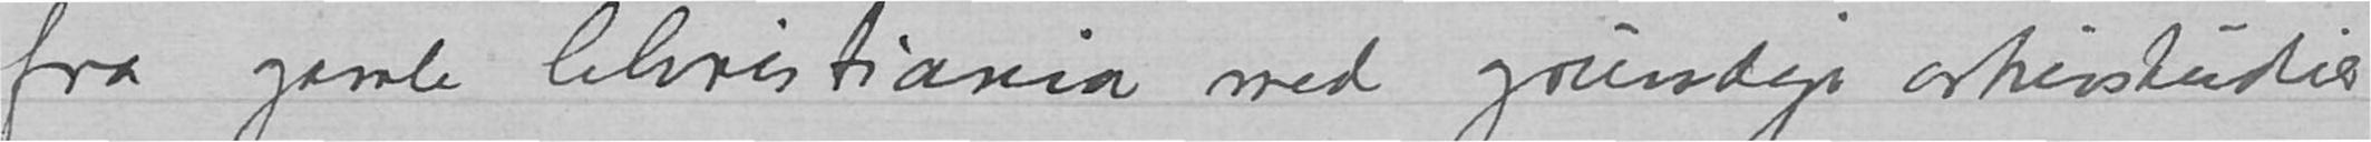fra gamle Christiania med grundige  arkivstudier
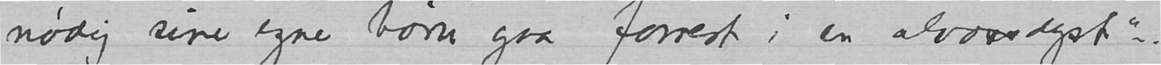nødig sine egne børn gaa forrest i en alvorsdyst»-.
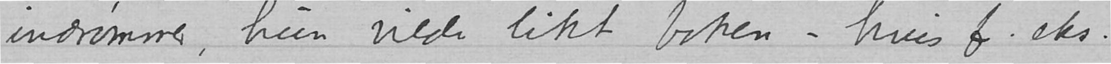indrømmer, hun vilde likt boken – hvis f. eks.
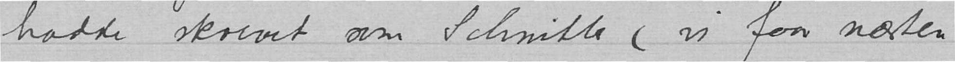hadde skrevet som Schnitler (vi faar næsten
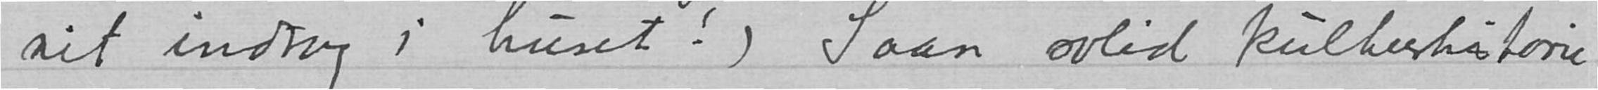sit indtog i huset!) Saan solid kulturhistorie
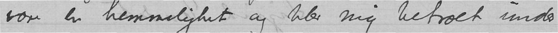være en hemmelighet og blev mig betroet under
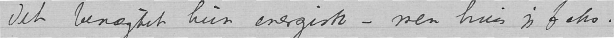Det benægtet hun energisk – men hvis jeg f eks.
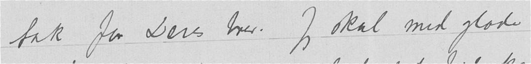tak for Deres brev. Jeg skal med glæde
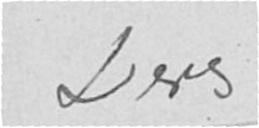Deres
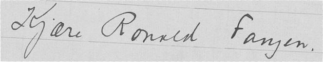Kjære Ronald Fangen,
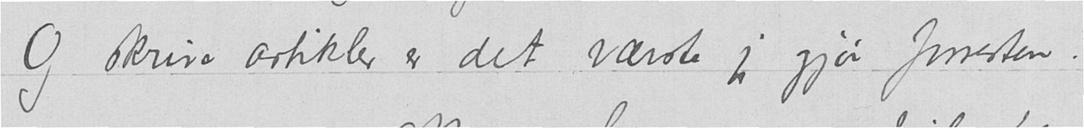Og skrive artikler er det værste jeg gjør forresten!
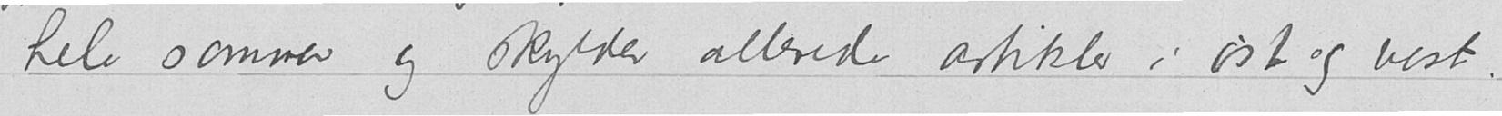hele sommer og skylder allerede artikler i øst og vest.
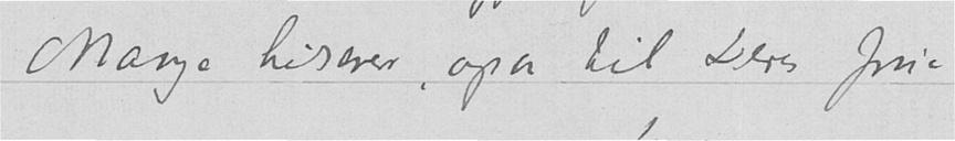Mange hilsener, ogsaa til Deres frue
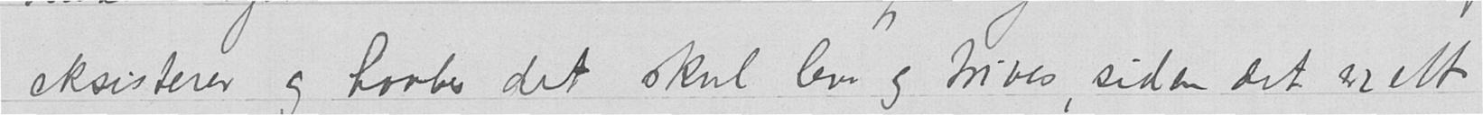eksisterer og haaber det skal leve og trives, siden det er ett
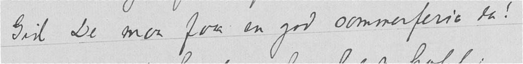Gid De maa faa en god sommerferie da!
